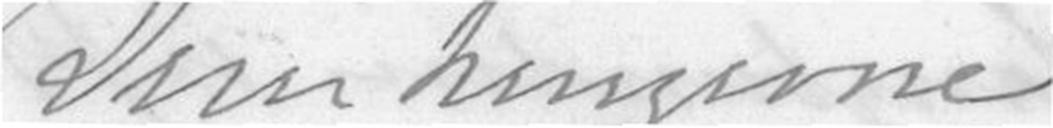Deres hengivne
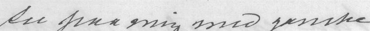ser paa mig med ganske
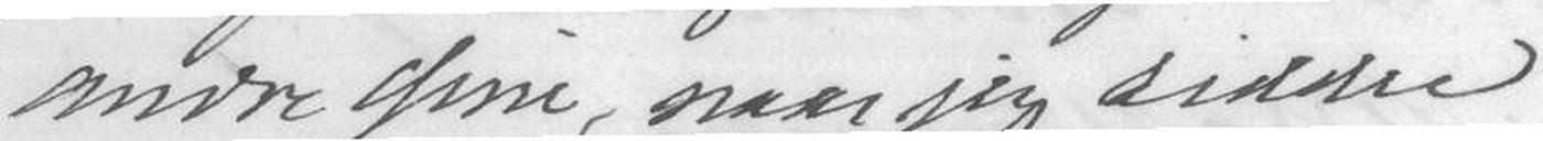andre Øine, naar jeg sidder
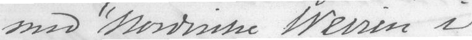med "Nordische Weisen" i
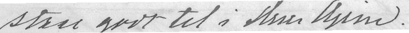staar godt til i Deres Hjem.
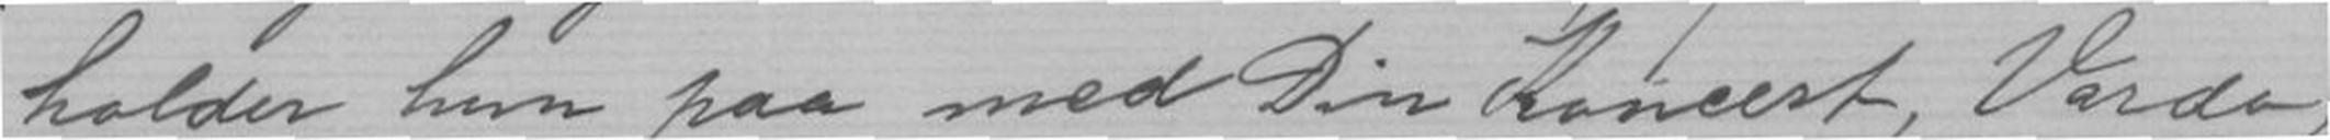holder hun paa med Din Koesert, Vardo,
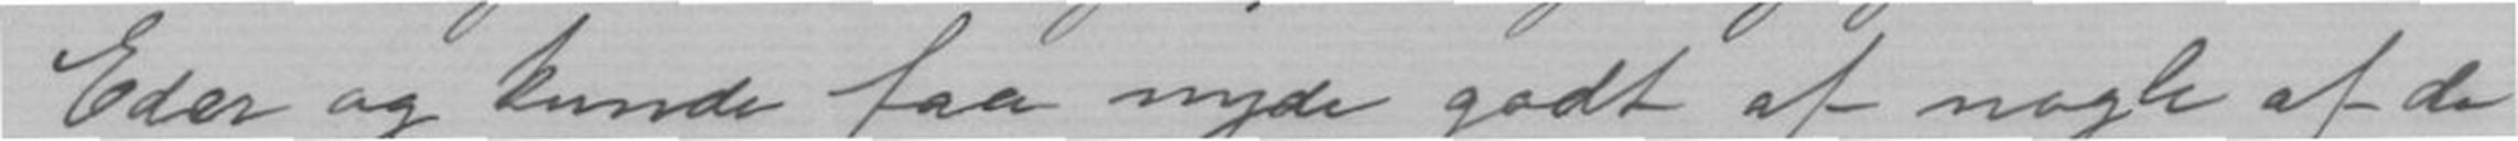Eder og kunde faa nyde godt af nogle af de
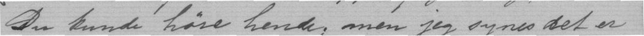Du kunde høre hende; men jeg synes det er
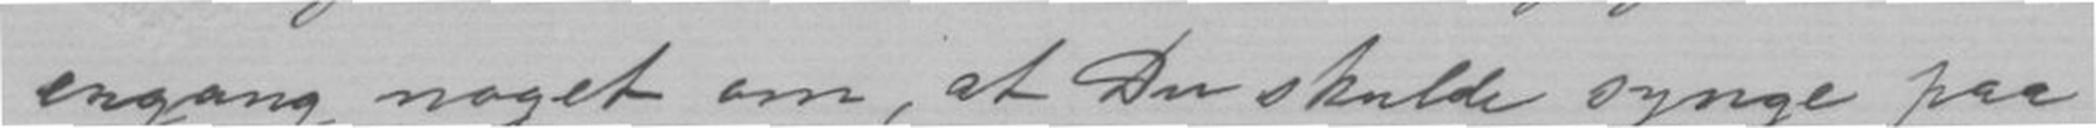engang noget om, at Du skulde synge paa
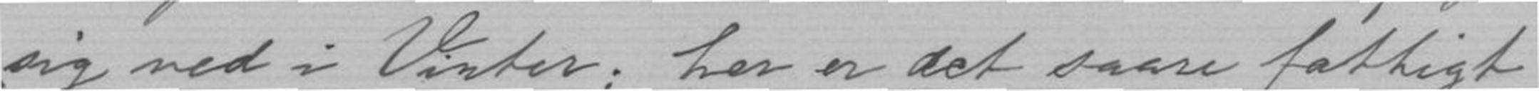sig ved i Vinter; her er det saare fattigt
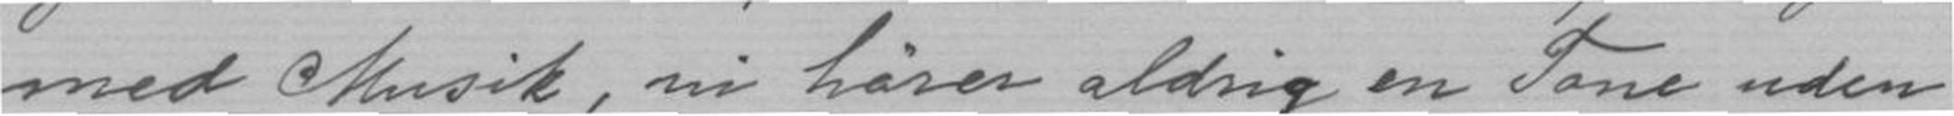med Musik, vi hører aldrig en Tone uden
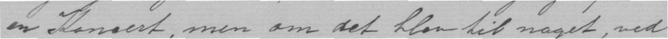en Konsert, men om det blev til noget, ved
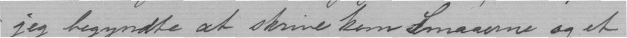jeg begyndte at skrive kom Smaaerne og et
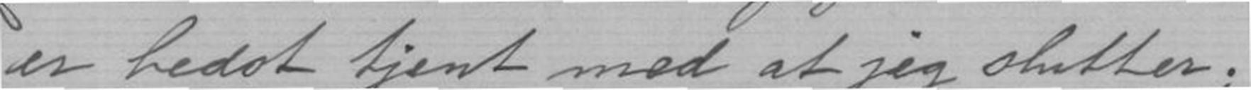er bedst tjent med at jeg slutter;
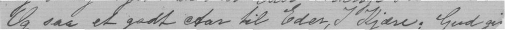Og saa et godt Aar til Eder, I Kjære; Gud gi
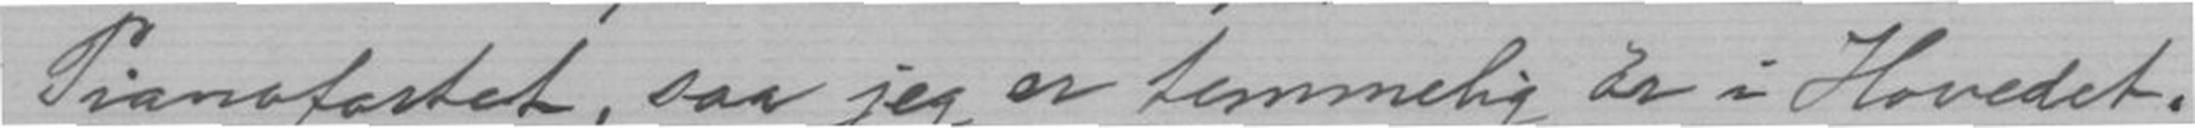Pianofortet, saa jeg er temmelig ør i Hovedet.
